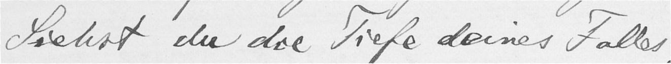Siehst du die Tiefe deines Falles,
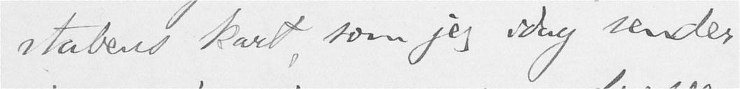stabens kart, som jeg idag sender
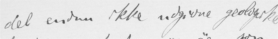del endnu ikke udgivne geologiske
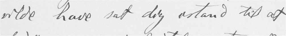vilde have sat dig istand til at
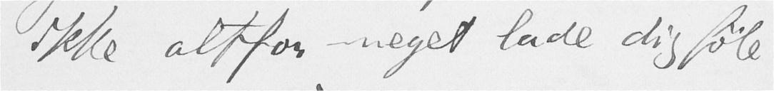ikke altfor meget lade dig føle
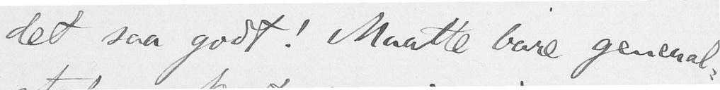det saa godt! Maatte bare general-
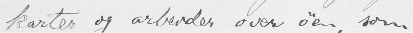karter og arbeider over øen, som
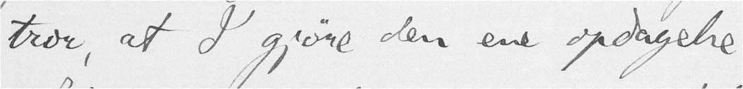tror, at I gjøre den ene opdagelse
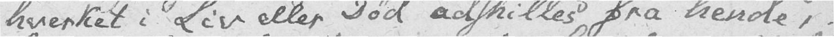hverket i Liv eller Død adskilles fra hende,
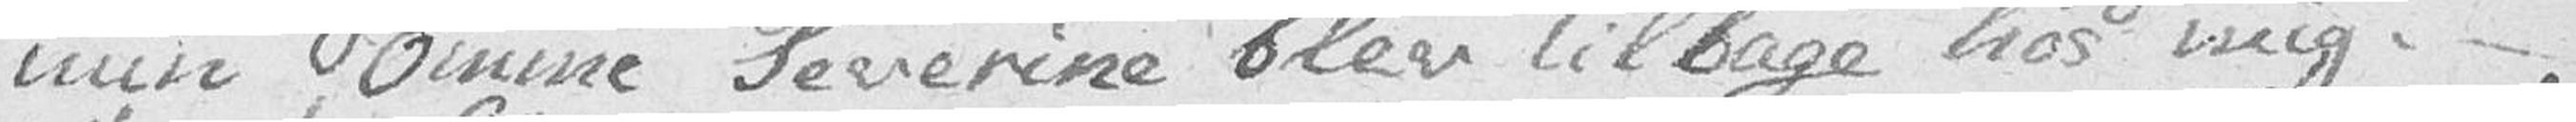min Ømme Severine blev tilbage hos mig. –
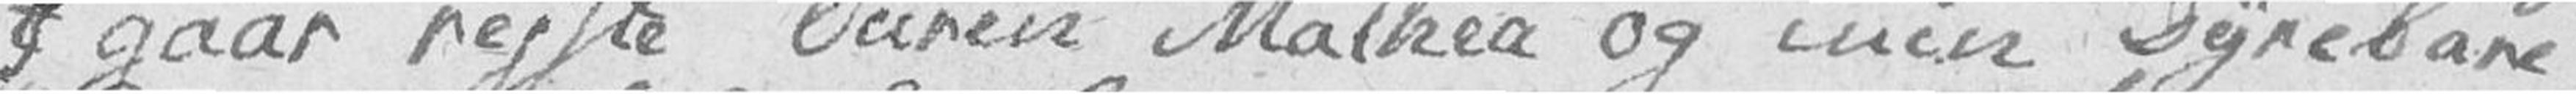I gaar reiste Ouren Mathea og min Dyrebare
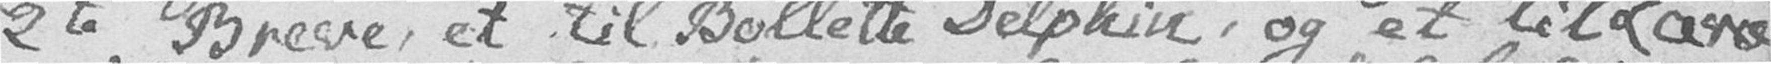2te Breve, et til Bollette Delphin, og et til Lasse
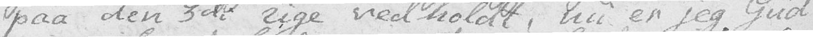paa den 3di Uge vedholdt, nu er jeg Gud
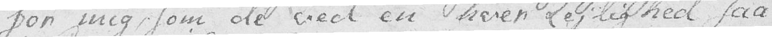for mig, som de ved en hver Lejlighed saa
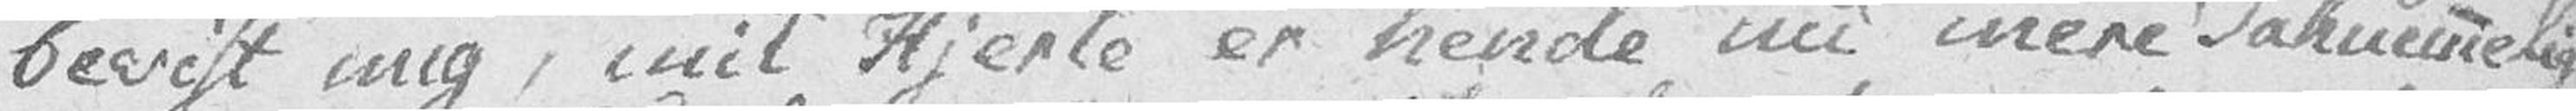bevist mig, mit Hjerte er hende nu mere Taknemmelig
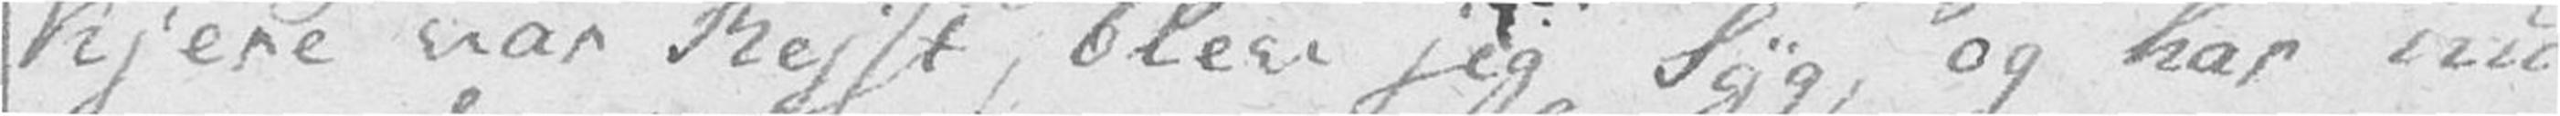kjere var Reist, blev jeg Syg, og har nu
